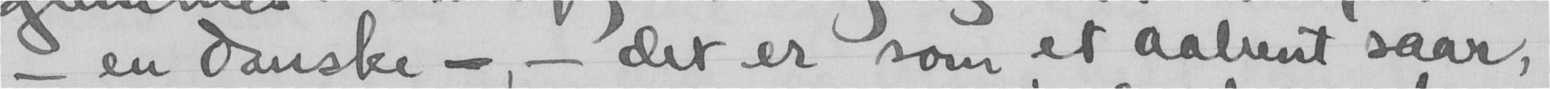- en danske -, - det er som et aabent saar,
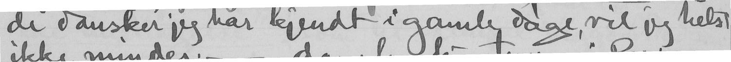de dansker jeg har kjendt i gamle dage, vil jeg helst
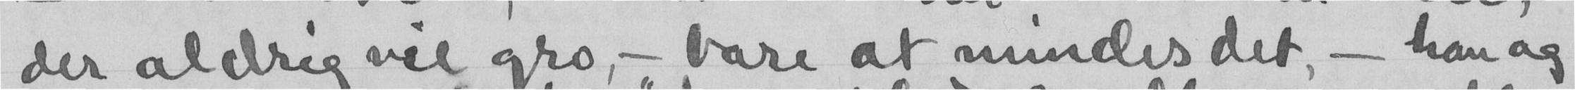der aldrig vil gro, - bare at mindes det, - han og
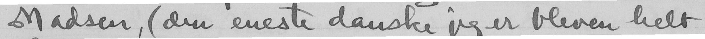Madsen, (den eneste danske jeg er bleven helt
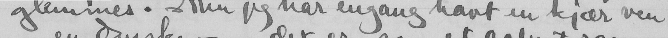glemmes. Men jeg har engang havt en kjær ven
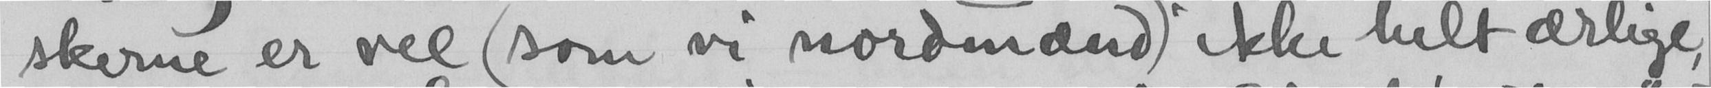skerne er vel (som vi nordmænd) ikke helt ærlige,
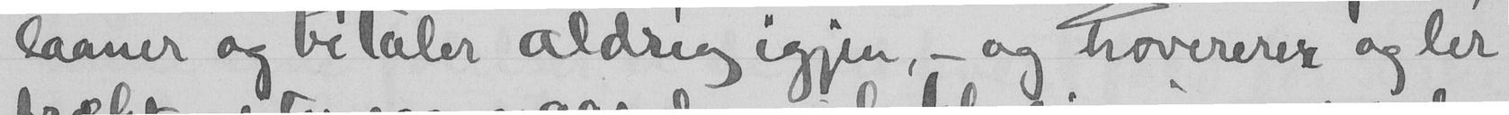laaner og betaler aldrig igjen, - og hovererer og ler
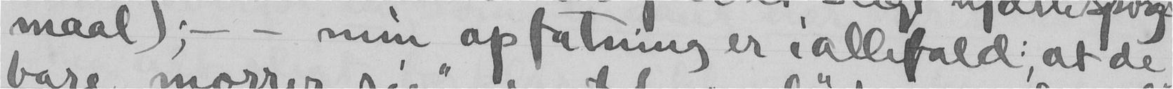maal); - min opfatning er iallefald:, at de
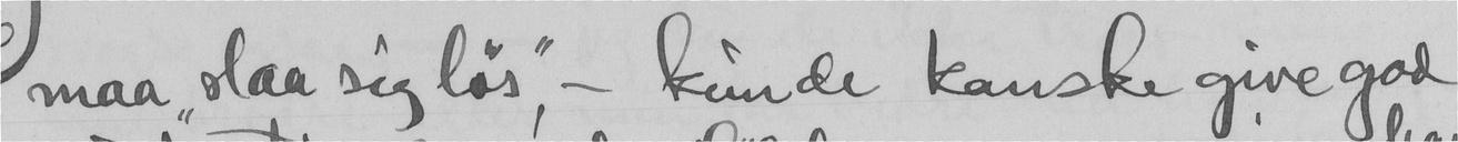maa slaa sig løs" - kunde kanske give god
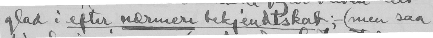glad i efter nærmere bekjendtskab; - (men saa
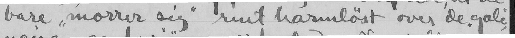bare "morrer sig" rent harmløst over de "gale",
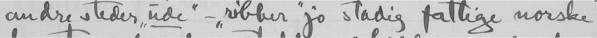andre steder "ude" - "ribber" jo stadig fattige norske
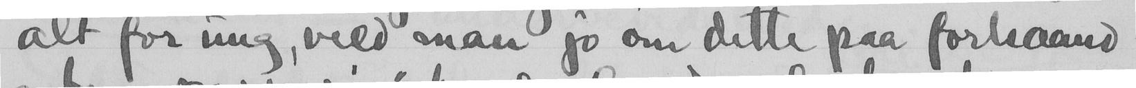alt for ung, veed man jo om dette paa forhaand
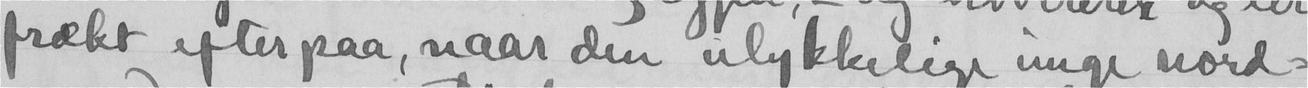frækt efter paa, naar den ulykkelige unge nord-
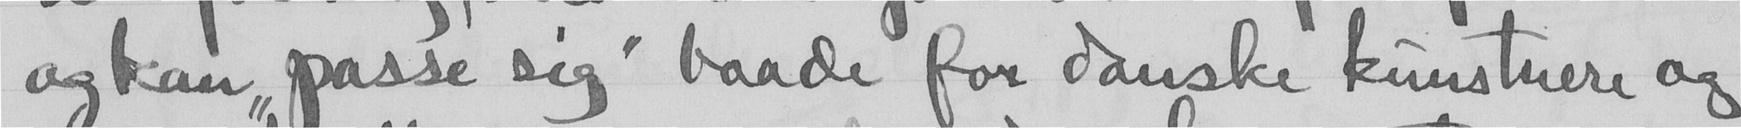og kan "passe sig" baade for danske kunstnere og
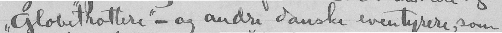"globetrottere" - og andre danske eventyrere, som
